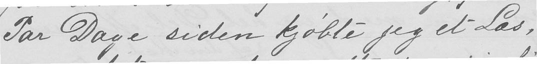Par Dage siden kjøbte jeg et Las,
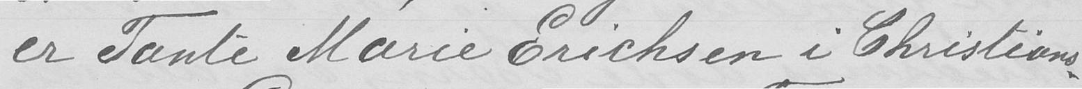er Tante Marie Erichsen i Christians-
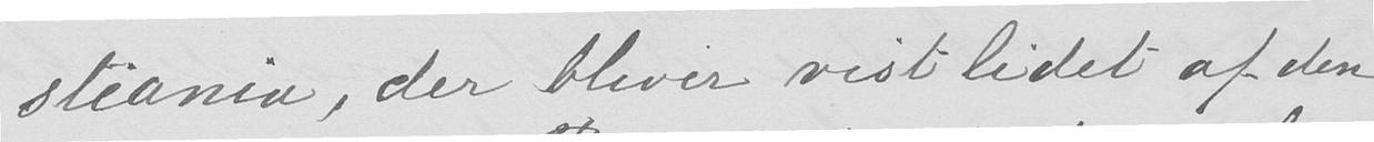stiania, der bliver vist lidet af den
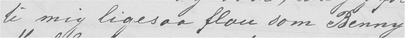te mig ligesaa flau som Benny
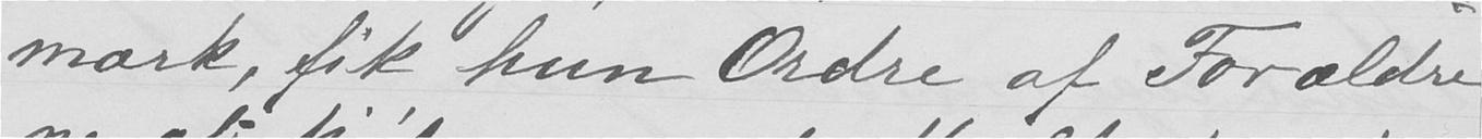mark, fik hun Ordre af Forældre-
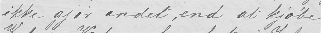ikke gjør andet, end at kjøbe
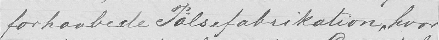forhaabede Pølsefabrikation, hvor-
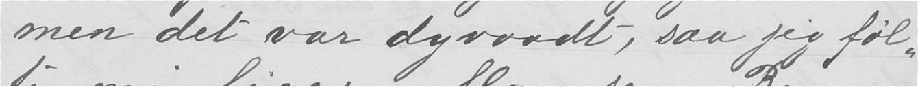men det var dyvaadt, saa jeg føl-
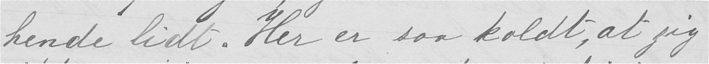hende lidt. Her er saa koldt, at jeg
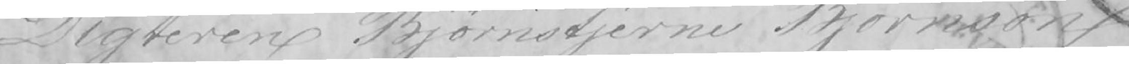Digteren Bjørnstjerne Bjørnson
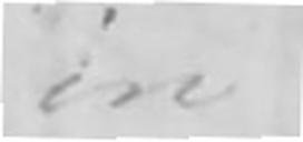in
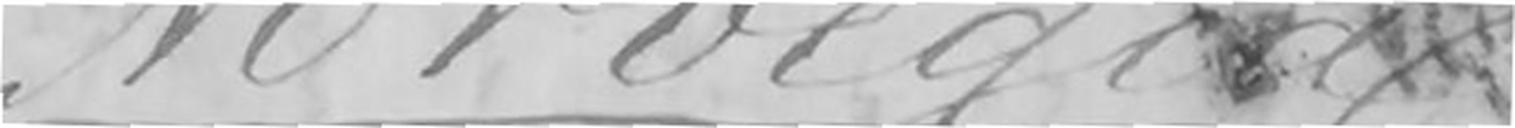Norvegia
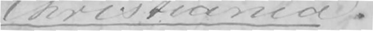Christiania
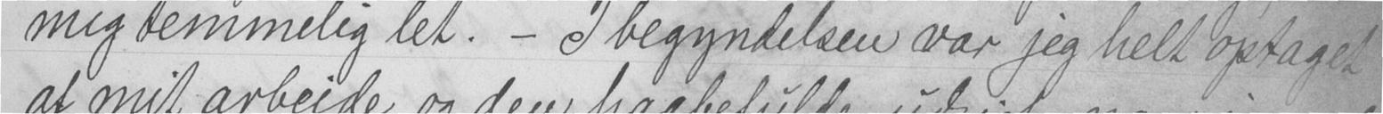mig temmelig let. - I begyndelsen var jeg helt optaget
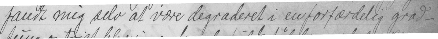fandt mig selv at være degraderet i en forfærdelig grad -
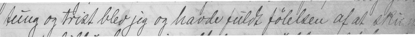tung og trist blev jeg og havde fuldt følelsen af at sku
